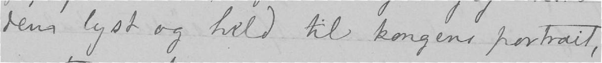dem lyst og held til kongens portrait,
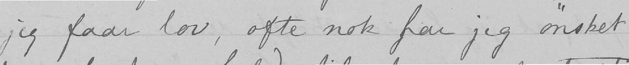jeg faar lov, ofte nok har jeg ønsket
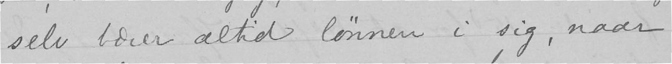selv bærer altid lønnen i sig, naar
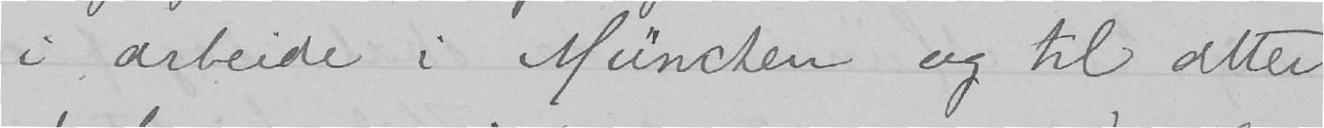i arbeide i München og til atter
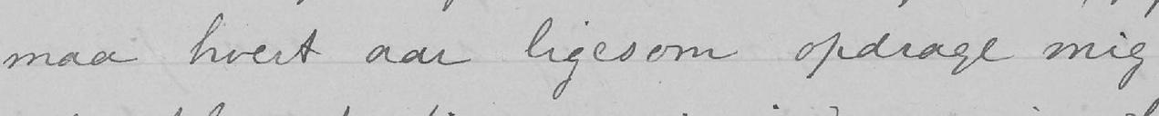maa hvert aar ligesom opdrage mig
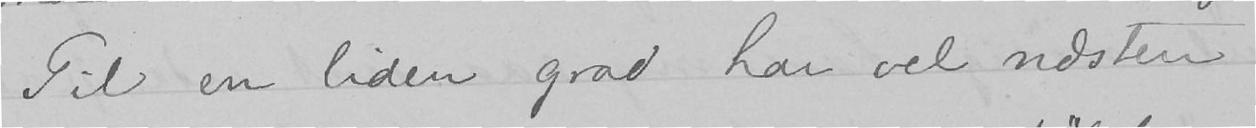Til en liden grad har vel næsten
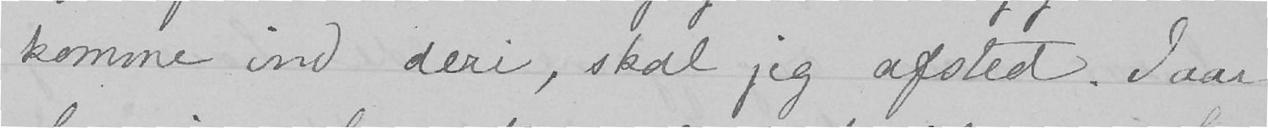komme ind deri, skal jeg afsted. Iaar
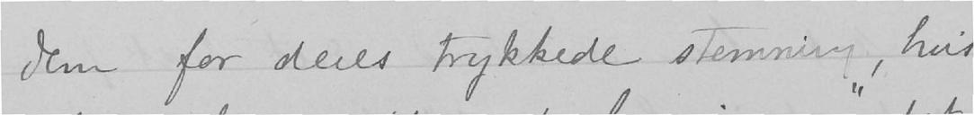dem for deres trykkede stemning, hvis
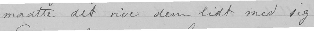maatte det rive dem lidt med sig.
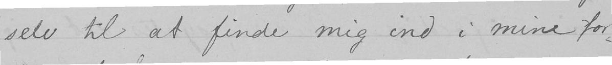selv til at finde mig ind i mine for-
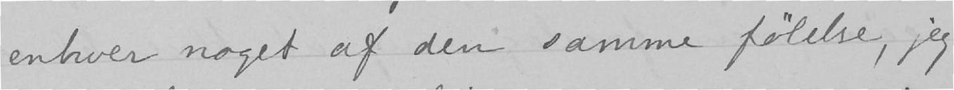enhver noget af den samme følelse, jeg
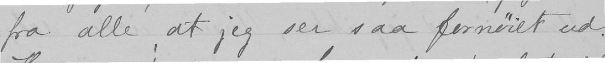fra alle, at jeg ser saa fornøiet ud.
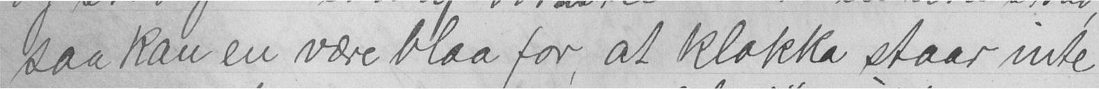saa kan en være blaa for, at klokka staar inte
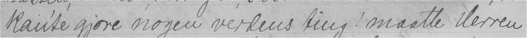kan'te gjøre nogen verdens ting! maatte Herren
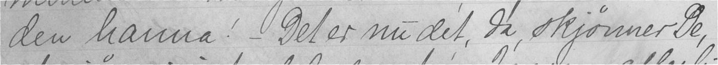den hanna! - Det er nu det, da, skjønner De,
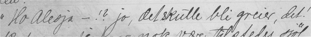"Ho Alesja - !? jo, det skulle bli greier, det!
